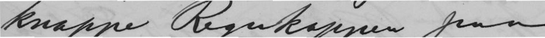knappe Regnkappen paa
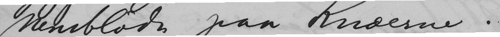nemblød paa Knæerne.
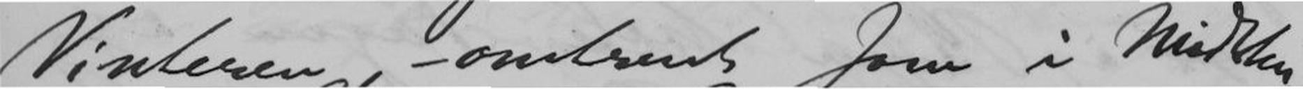Vinteren, - omtrent som i Midten
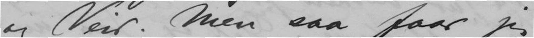og Veir. Men saa faar jeg
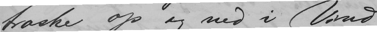traske op og ned i Vind
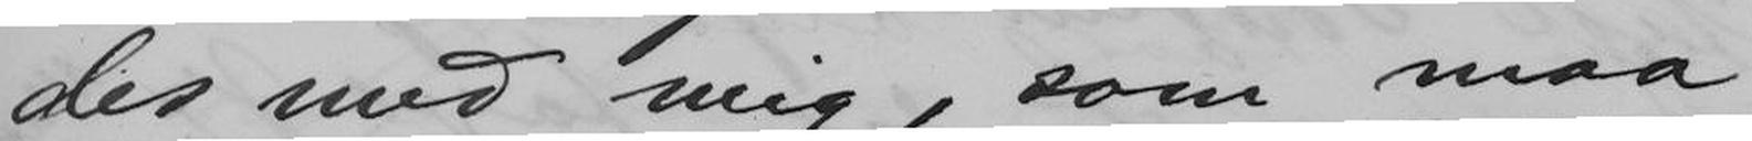des med mig, som maa
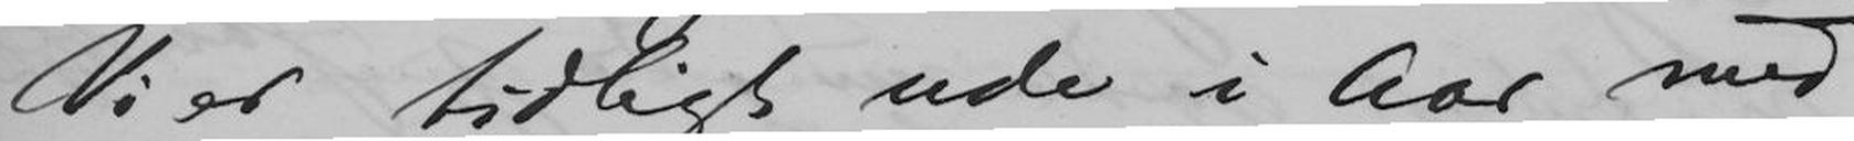Vi er tidligt ude i Aar med
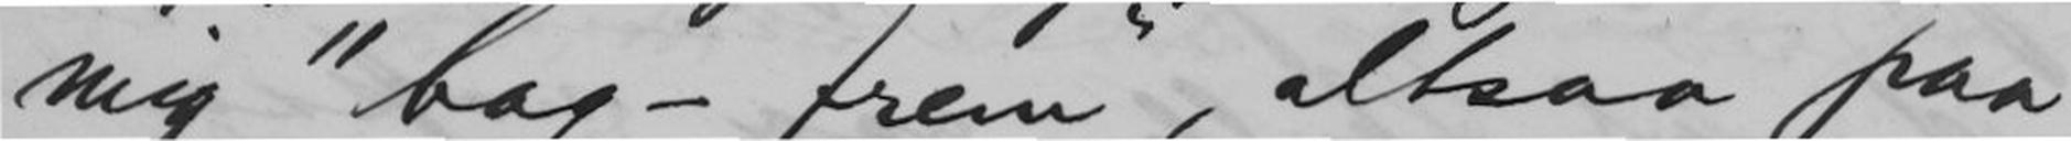mig "bag-frem", altsaa paa
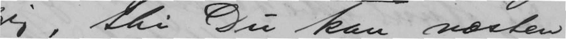zig, thi Du kan næsten
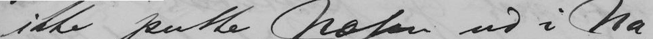ikke putte Næsen ud i Na-
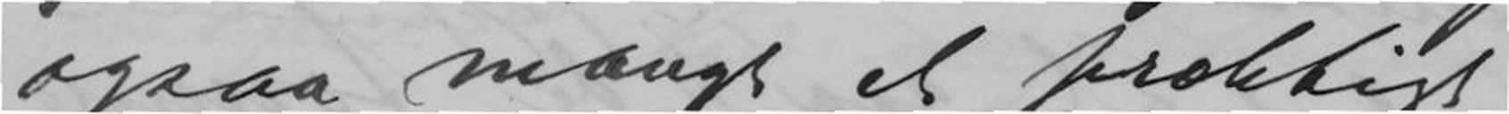ogsaa mangt et præktigt
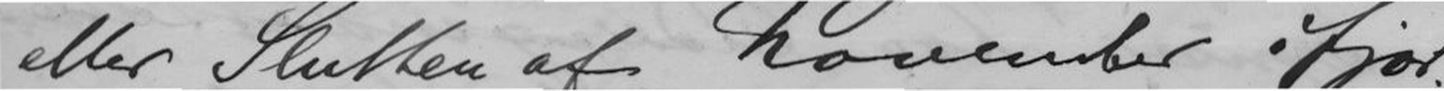eller Slutten af November ifjor.
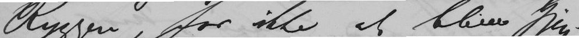Ryggen, for ikke at blive gjen-
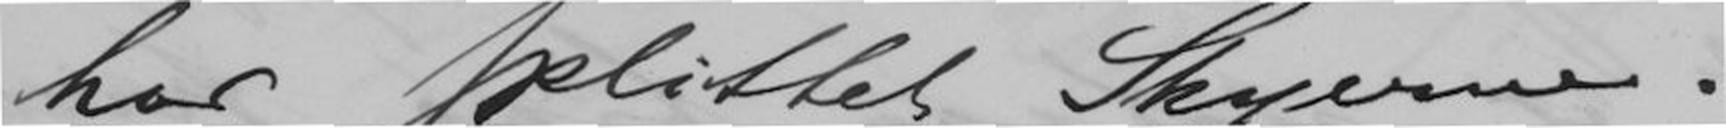har splittet Skyerne.
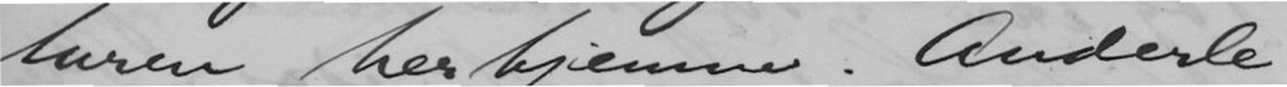turen her hjemme. Anderle-
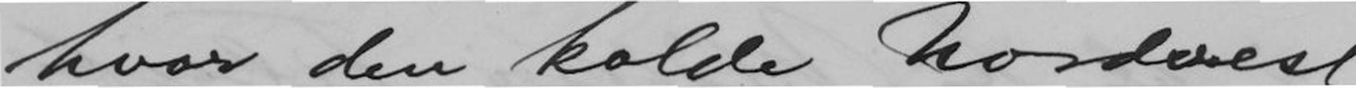hvor den kolde Nordvest
